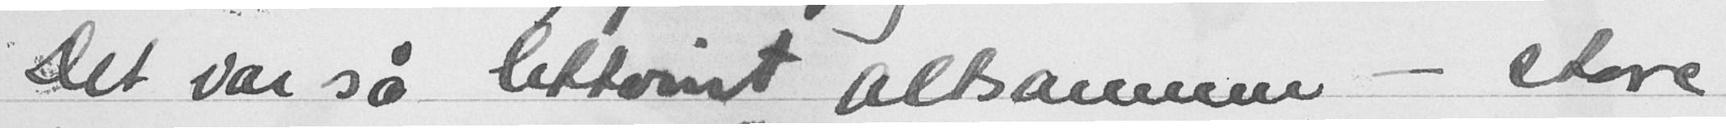Det var så lettvint altsammen - store
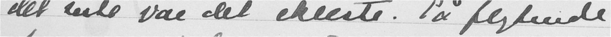det siste var det ekleste. På flytende
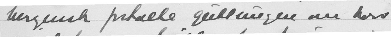bergensk fortalte guttungen om hvor
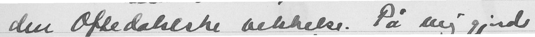den Oftedahlske vekkelse. På mig gjorde
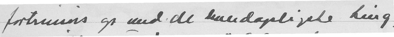fortrinnsvis op med de hverdagsligste ting,
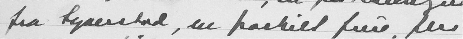fra tyserstad, en fraskilt frue, fler
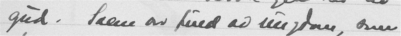gud. Salen var fuld av ungdom, som
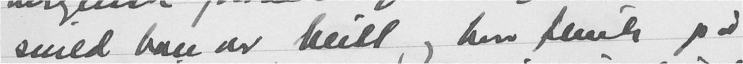snild han var blitt og hvor flink på
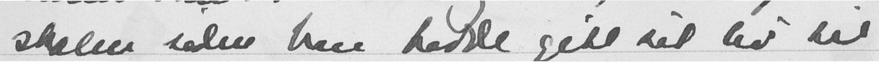skolen siden han hadde gitt sit liv til
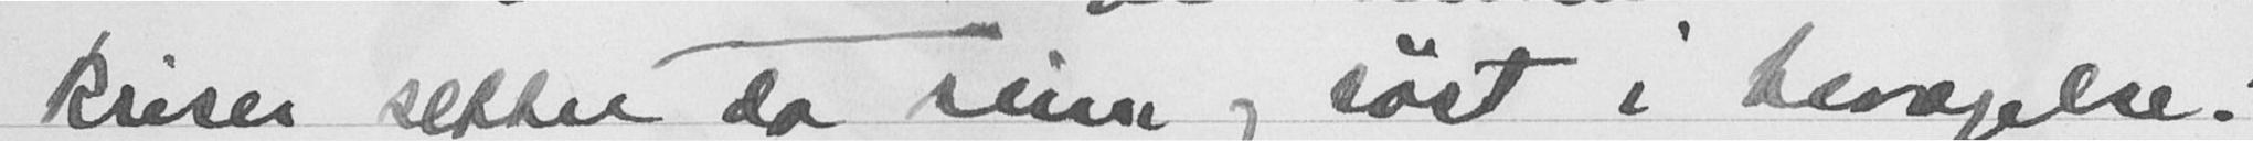kriser setter da sinn og røst i bevægelse!
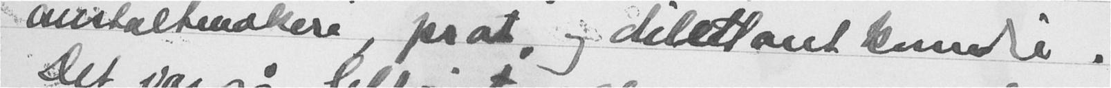anstaltmakere, prat, og dillitant kvindre.
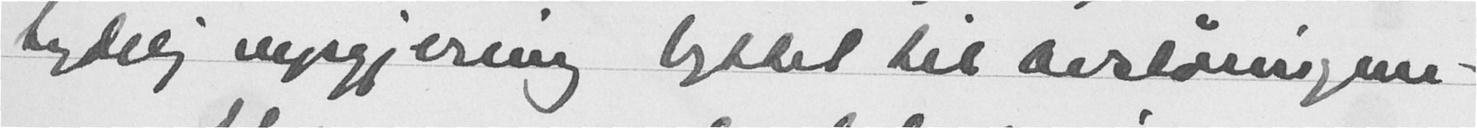tydelig nysgjerrig lyttet til avsløringene.
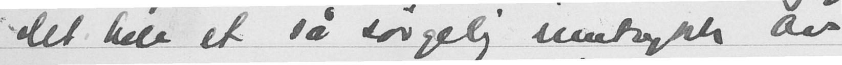det hele et så sørgelig inntrykk av
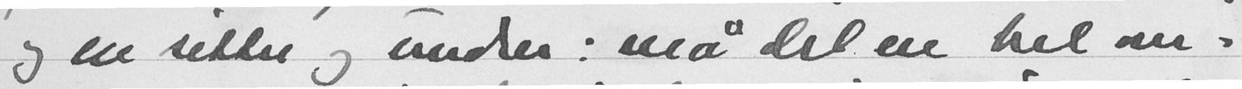og en sitter og undrer; må det en helom-
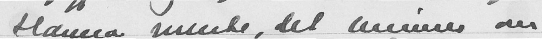Hanna mente, det minner om
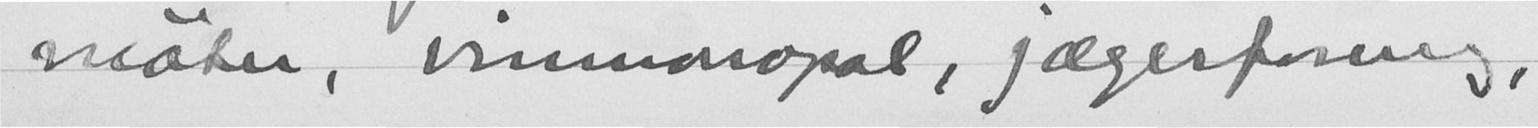møter, Vinmonopol, jægerforening,
